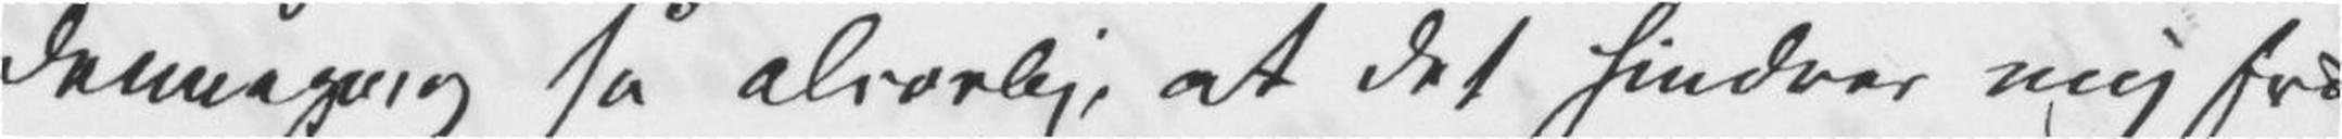dennegang så alvorlig, at det hindrer mig fra
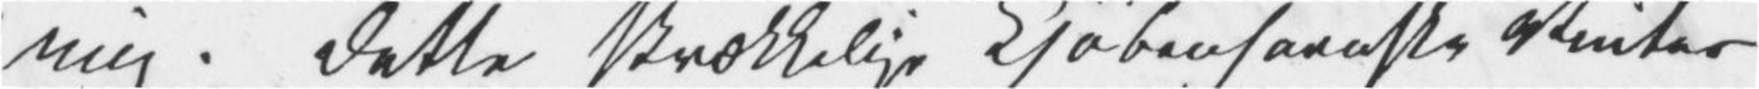mig. Dette skrækkelige Kjøbenhavnske Vinter¬
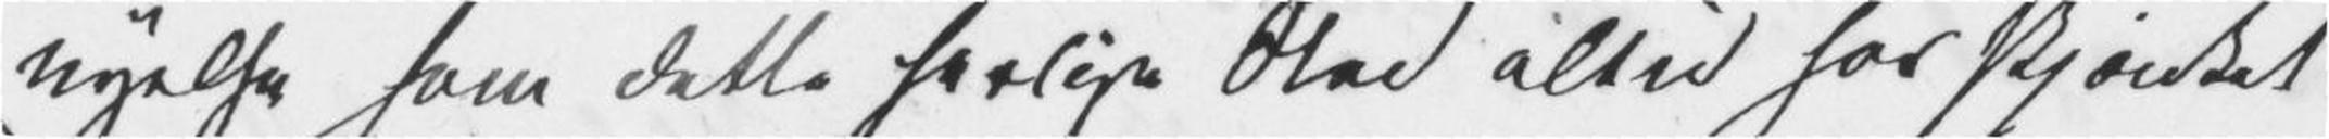nyelse som dette herlige Sted altid har skjænket
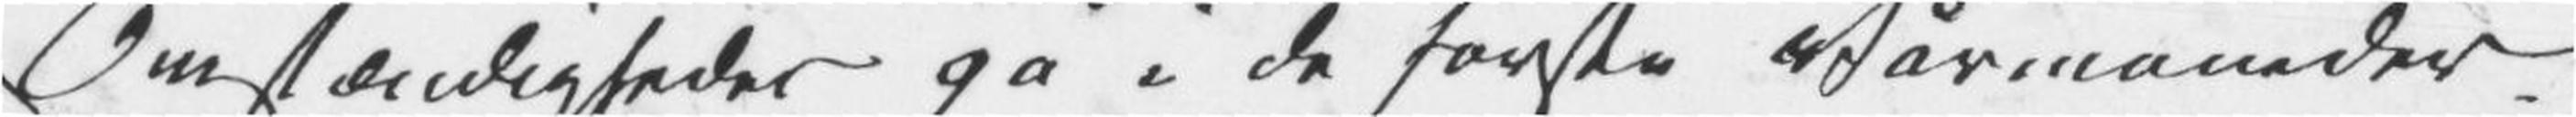Omstændigheder gå i de første Vårmåneder
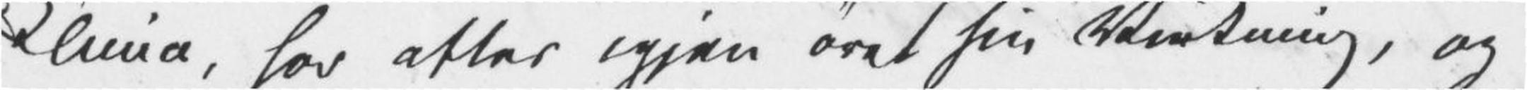klima, har atter igjen øvet sin Virkning, og
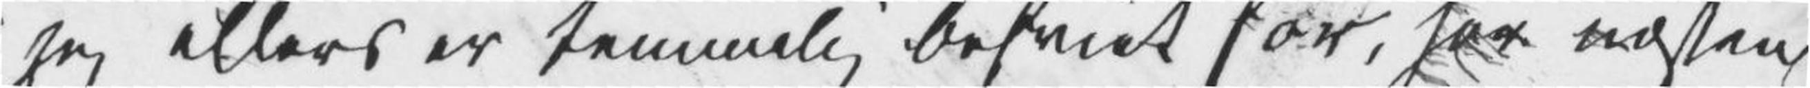jeg ellers er temmelig befriet for, har næsten
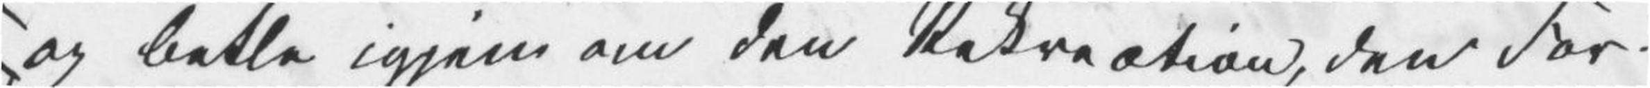og betle igjen om den Rekreation, den For¬
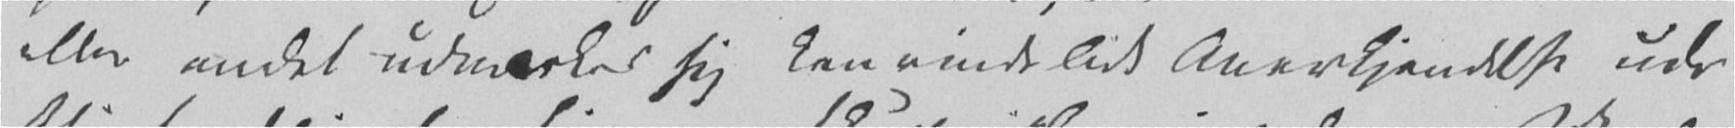eller andet udmærker sig kan vinde lidt Anerkjendelse ude
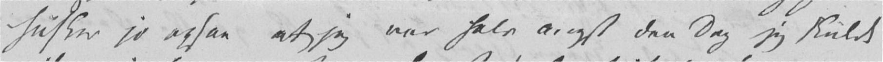husker jo ogsaa at jeg var selv angst den Dag jeg skulde
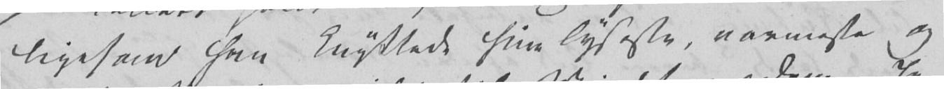ligesom han knyttede sine lyseste, varmeste og
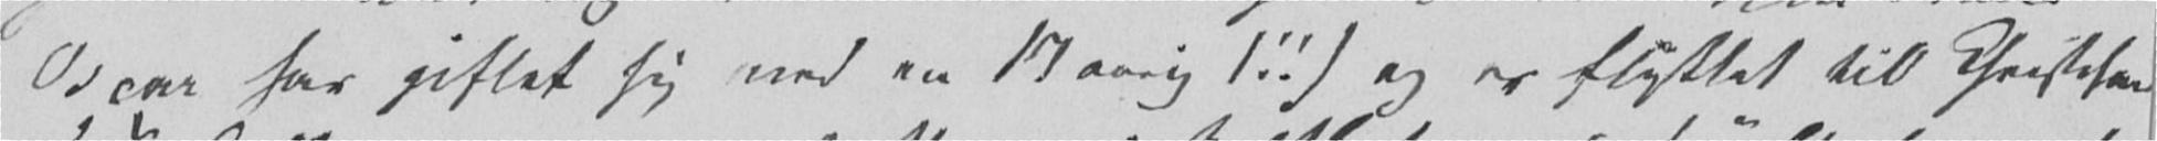Oscar har giftet sig med en 17 aarig (!!) og er flyttet til Christisan
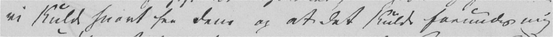vi skulde snart see Dem og at det skulde forundes mig
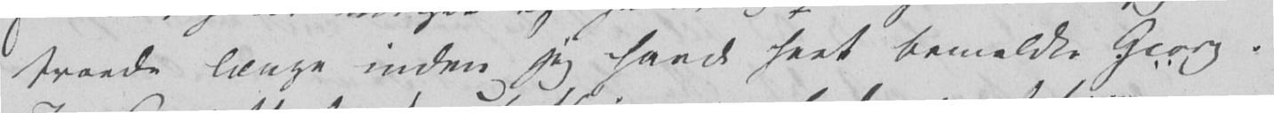troede længe inden jeg havde seet bemeldte Georg.
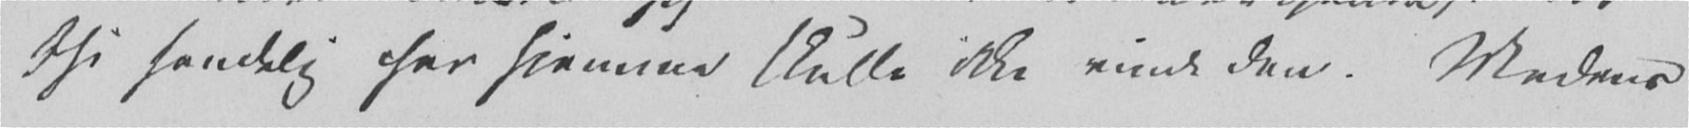thi sandelig her hjemme skulle ikke vinde den. Medens
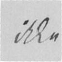ikke
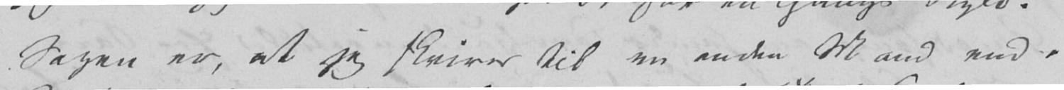Sagen er, at jeg skriver til en anden Mand end
